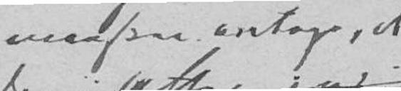maaskee antage, at
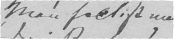Men factisk maa
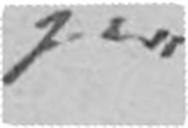ger.
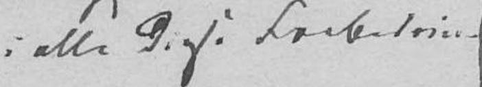i alle disse Forbedrin-
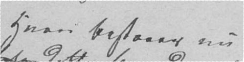Hvori bestaaer nu
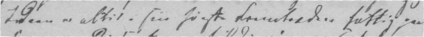Ideen er altid i sin første Fremtræden fattig paa
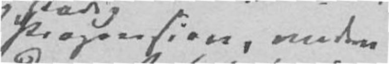Proporsion, medens
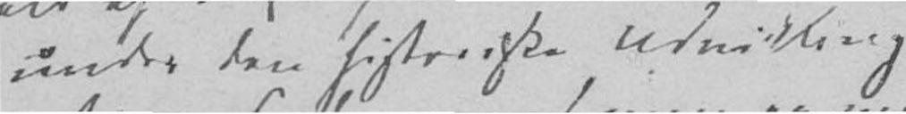under den historiske Udvikling
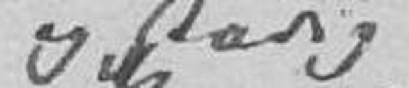og stadig
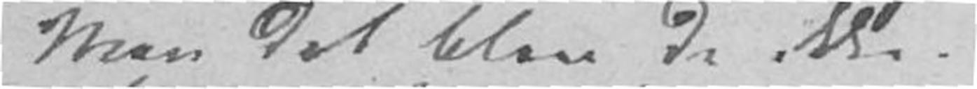Men det blev de ikke.
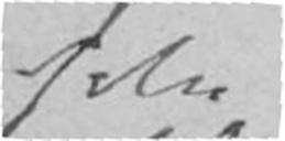selv
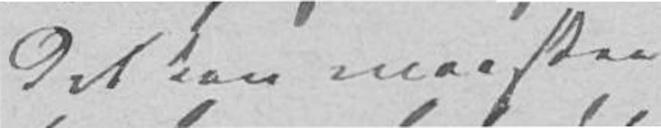Det kan maaskee
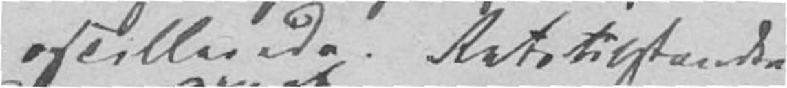oscillerede. Retstilstanden
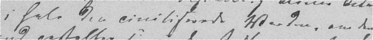i hele den civiliserede Verden, om den
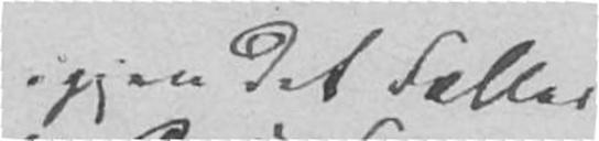igjen det Fælles
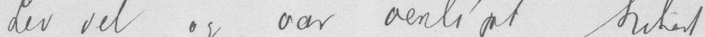Lev vel og vær venligt
